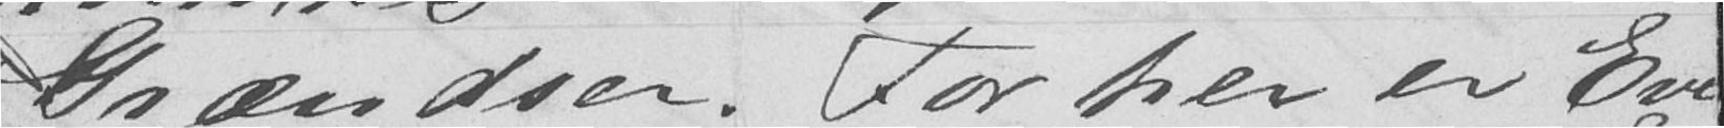Grændser. For her er Eve
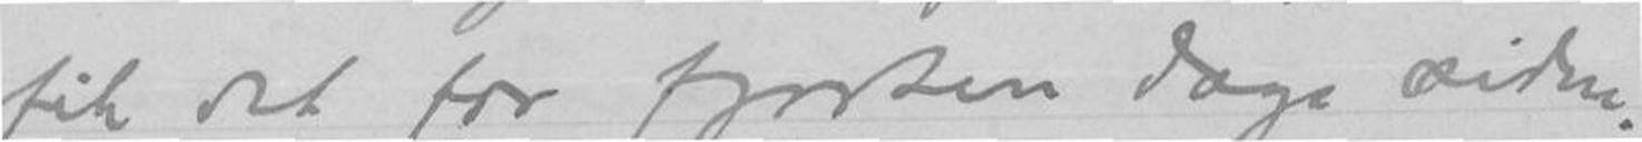fik det for fjorten dage siden.
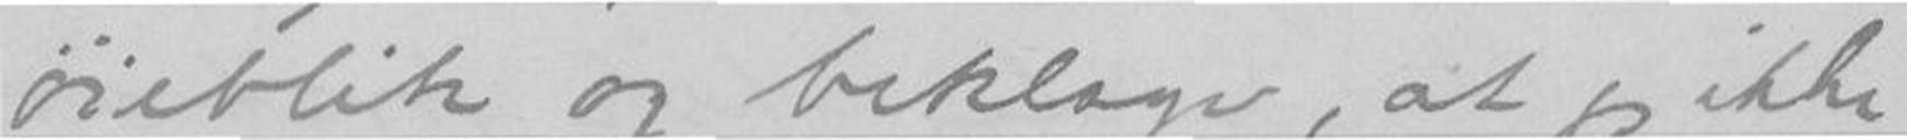øieblik og beklager, at jeg ikke
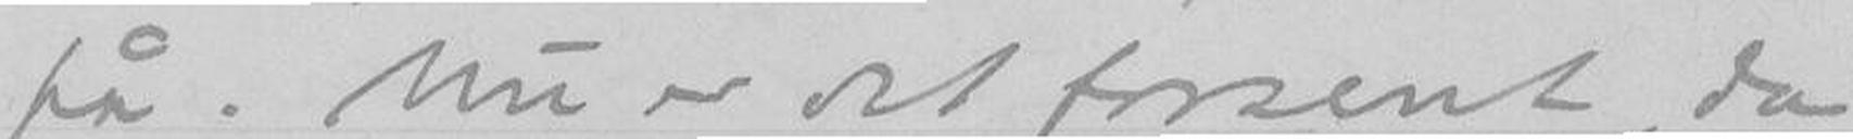på. Nu er det forsent, da
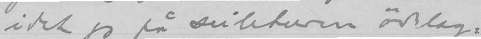idet jeg på seileturen ødelag-
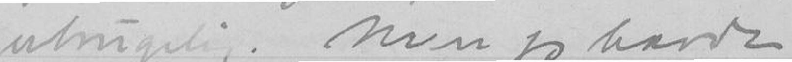ubrugelig. Men jeg havde
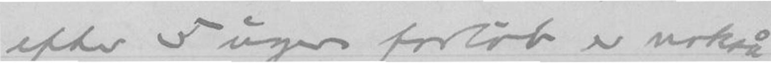efter 5 ukers forløb er nokså
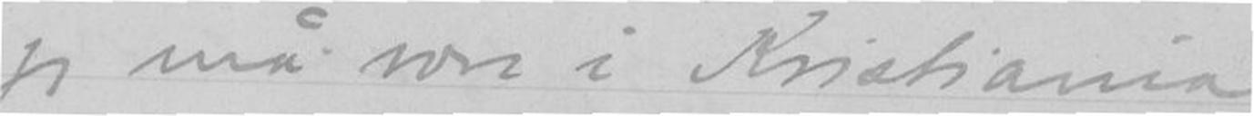jeg må være i Kristiania
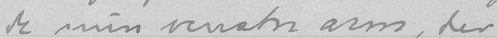de min venstre arm, der
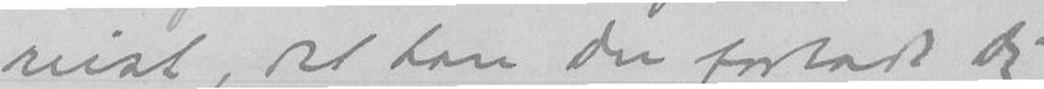reist, det kan du forlade deg
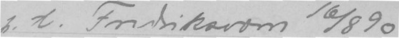p.t. Fredriksværn 16/8 90
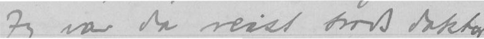Jeg var da reist trods doktor
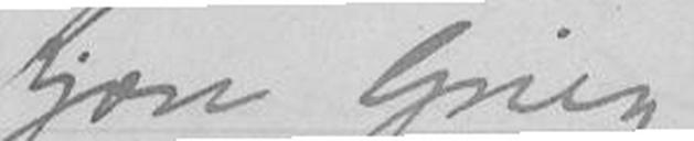Kjære Grieg
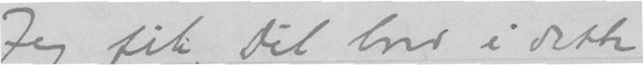Jeg fik dit brev i dette
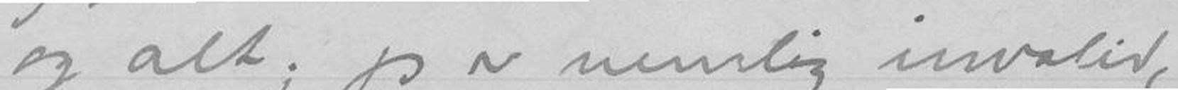og alt; jeg er nemlig invalid,
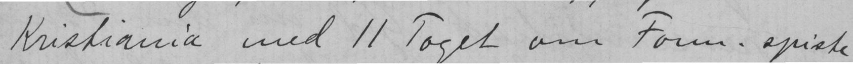Kristiania med 11 Toget om Form. spiste
